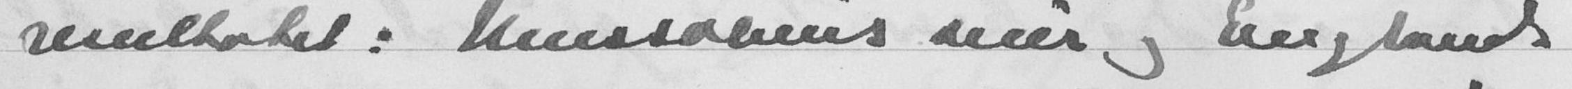resultatet: Mussolinis seier og Englands
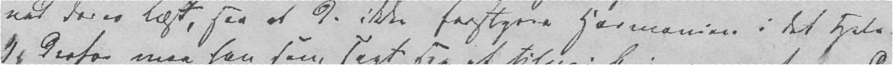ved deres Læst, saa at de ikke forstyrre Harmonien i det Hele.
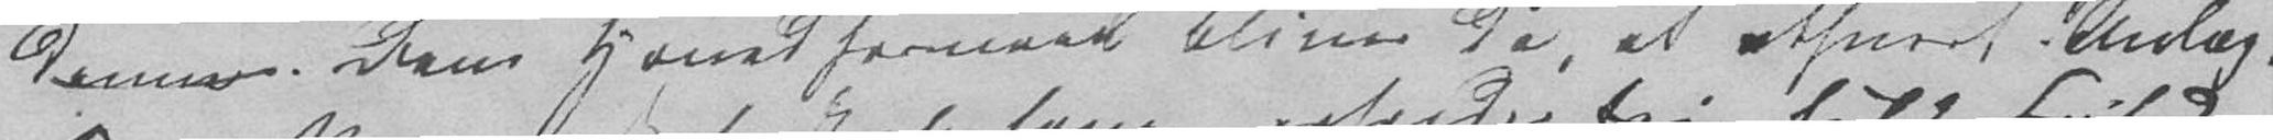dannes. Dens Hovedformaal bliver da, at ethvert Anlæg
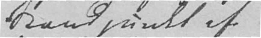Standpunkt af
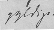gyldige.
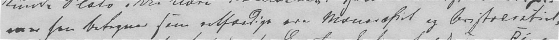mer han betegner som retfærdige ere Monarchiet og Aristocratiet,
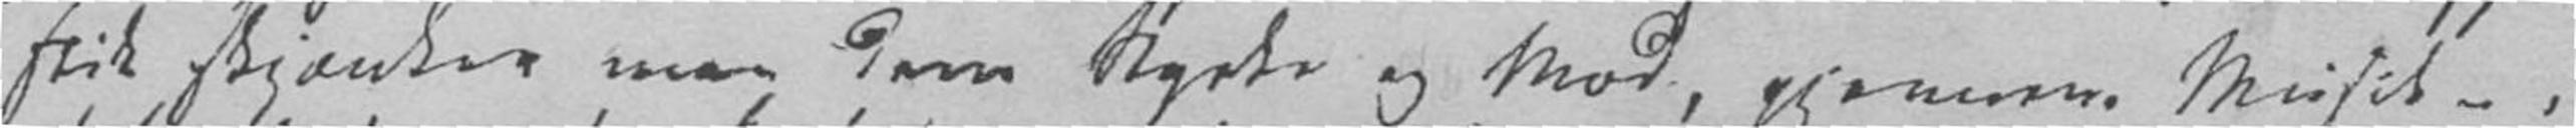stik skjænker man dem Styrke og Mod, gjennem Musik – i
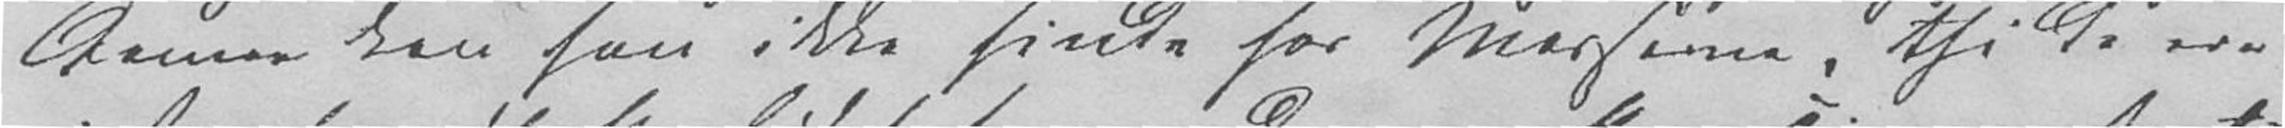Denne kan han ikke finde hos Masserne, thi de ere
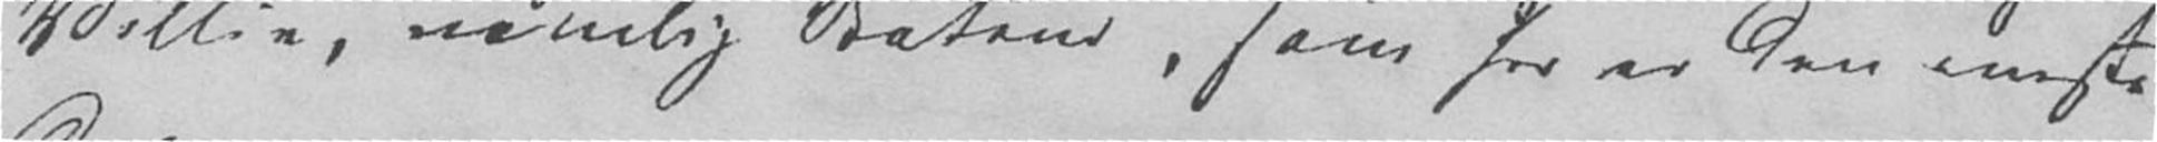Villie, nemlig Statens, som her er den eneste
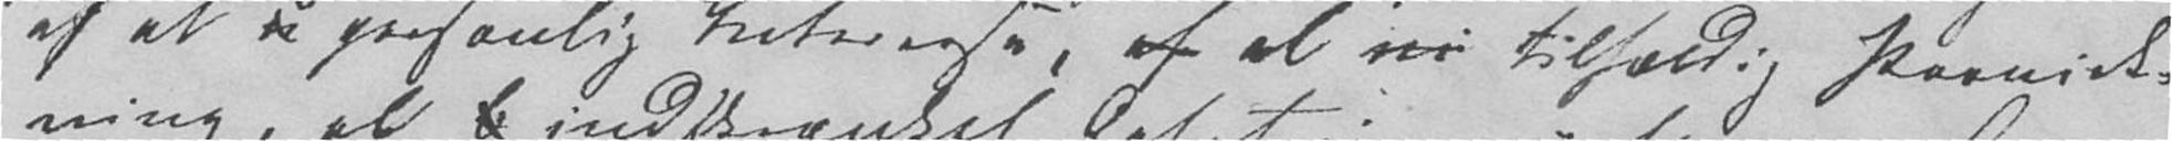af al i personlig Interesse, af al ni tilfældig Paavirk-
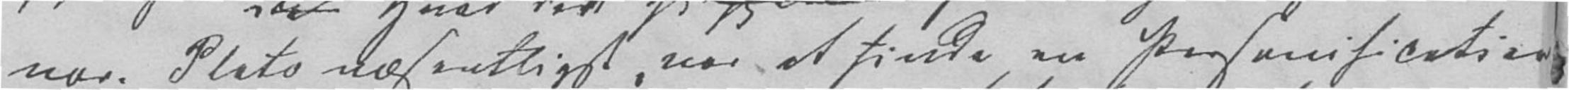være Plato væsentligst, var at finde en Personification
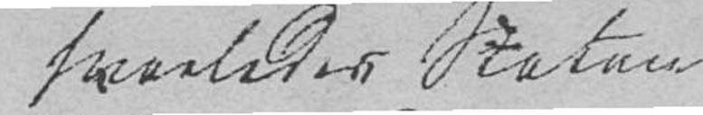hvorledes Staten
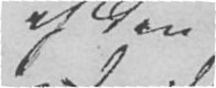af den
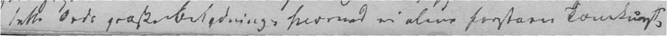dette Ords græske Betydning – hvorved ei alene forstaaes Tonekunst,
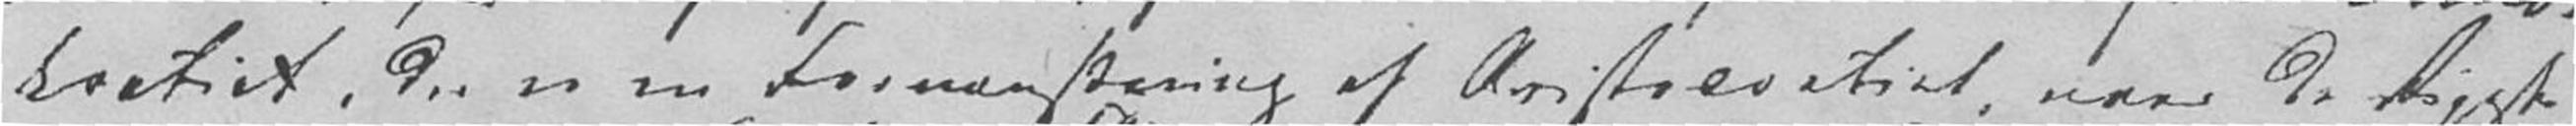cratiet, der er en Forvanskning af Aristocratiet, naar de Rigeste
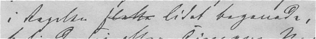i Regelen slette lidet begavede,
